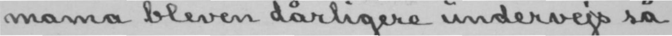mama bleven dårligere undervejs så
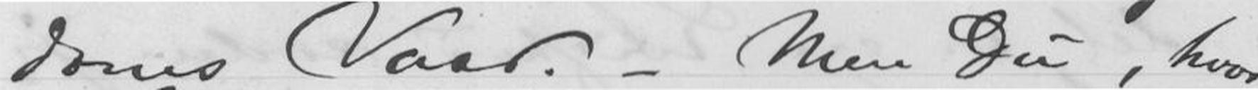doms Vaar. - Men Du, hvor
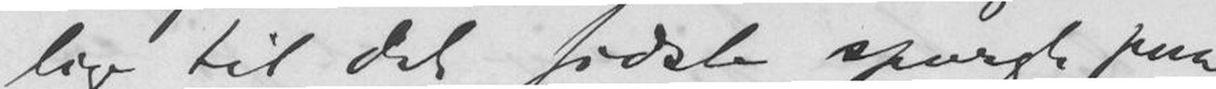lige til det sidste spurt paa
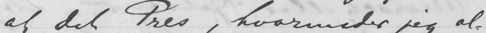at det Pres, hvorunder jeg al-
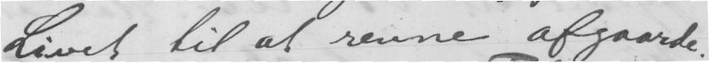Livet til at renne afgaarde.
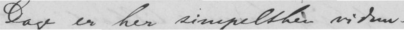Dage er her simpelthen vidun-
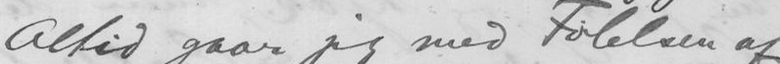Altid gaar jeg med Følelsen af
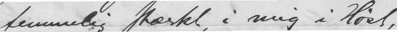temmelig stærkt i mig i Høst,
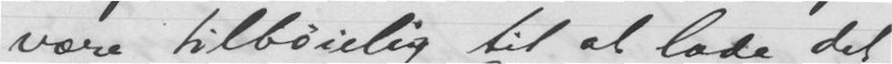være tilbøielig til at lade
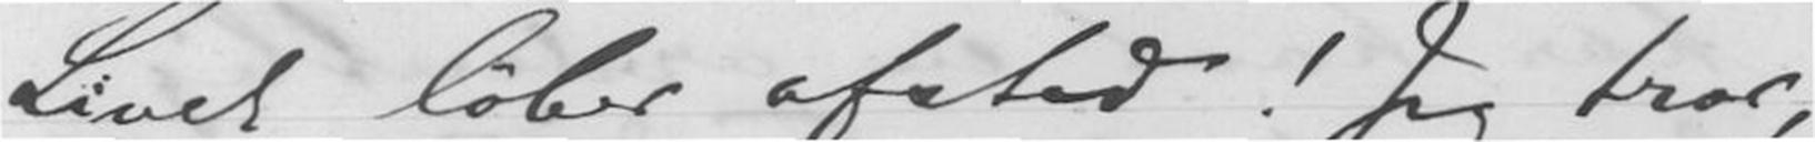Livet løber afsted! Jeg tror,
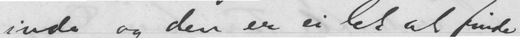inde og den er ei let at finde
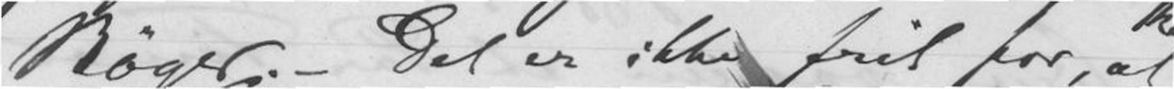Bøger. - Det er ikke frit for, at
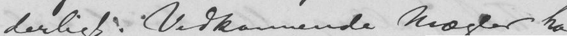derligt. Vedkommende Mægler har
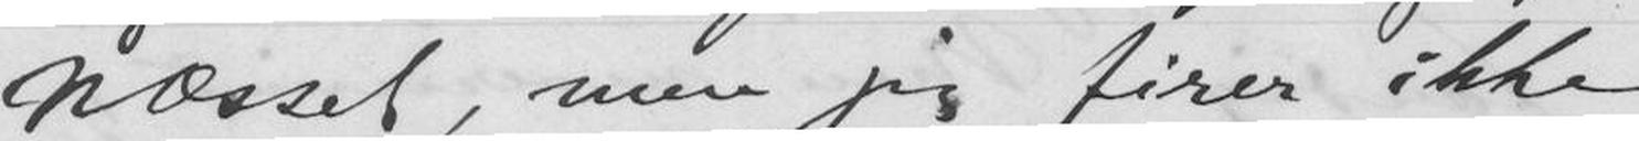Næsset, men jeg firer ikke
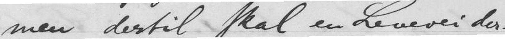men dertil skal en Levevei der-
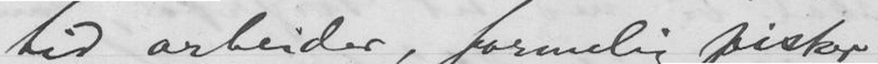tid arbeider, formelig pisker
